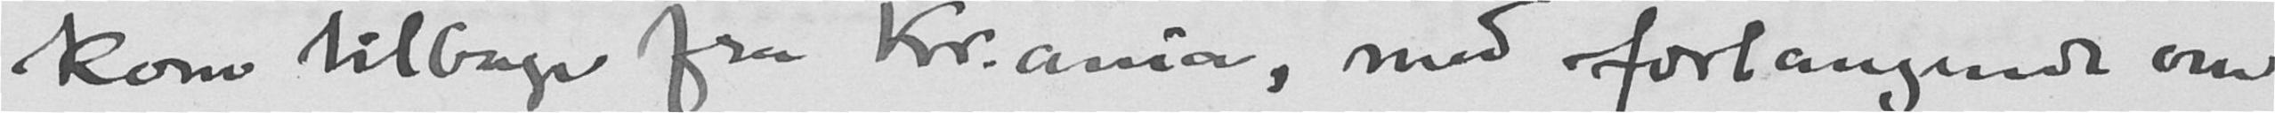kom tilbage fra Kr.ania, med forlangende om
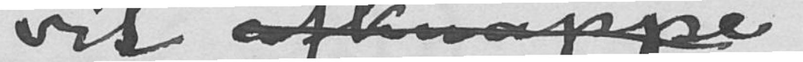vil afknappe
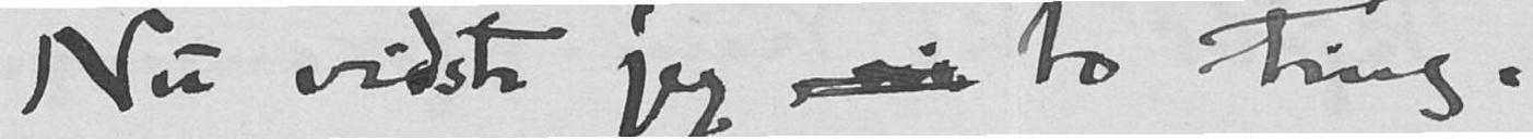Nu vidste jeg to ting.
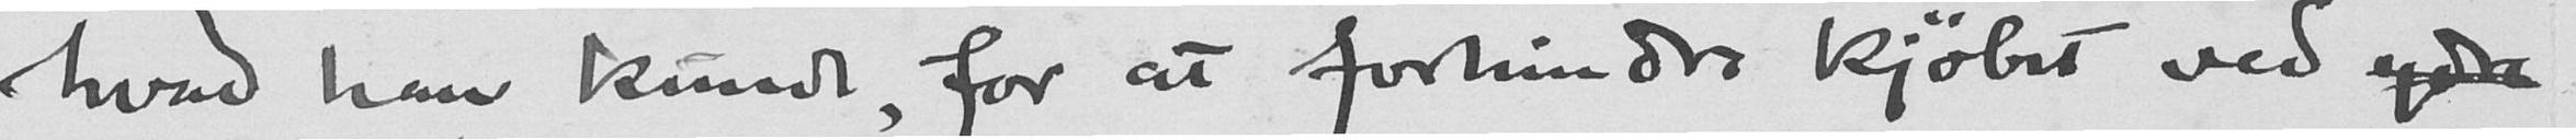hvad han kunde, for at forhindre kjøbet ved yder
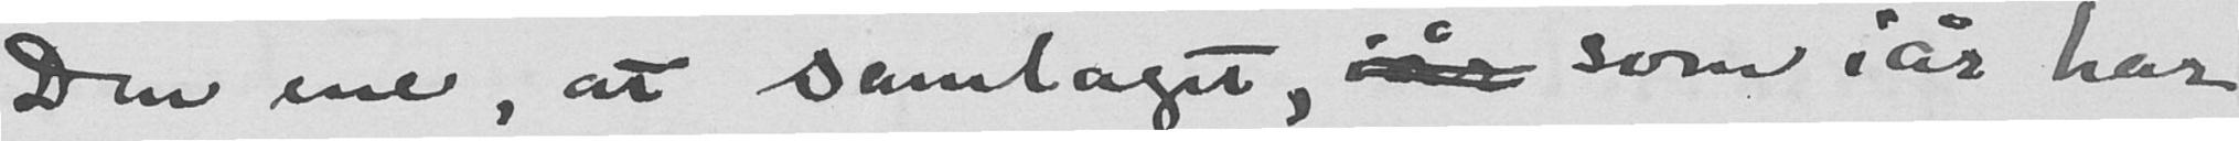Den ene, at samlaget, iår som iår har
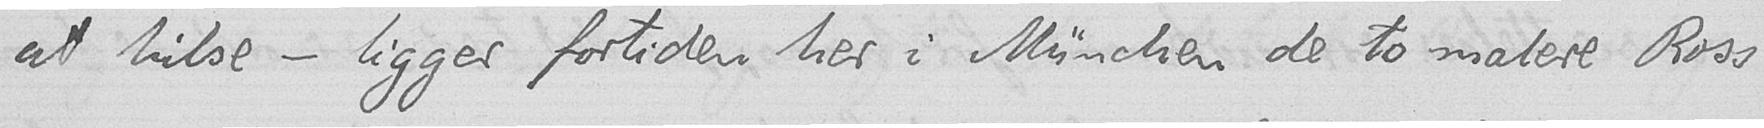at hilse - ligger fortiden her i München de to malere Ross
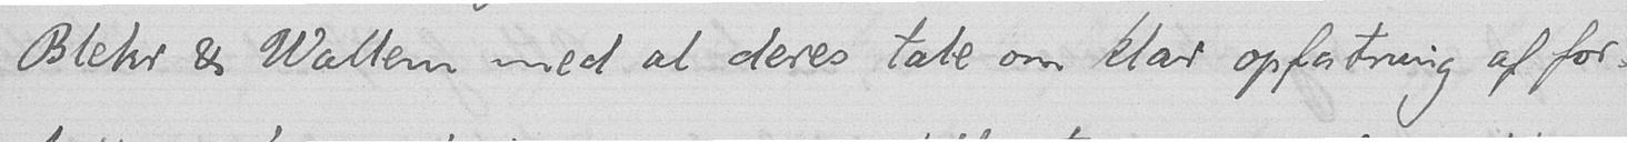Blehr & Wallem med al deres tale om klar opfatning af for-
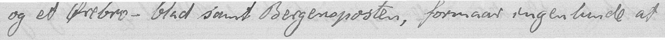og et Ørebro-blad samt Bergensposten, formaar ingenlunde at
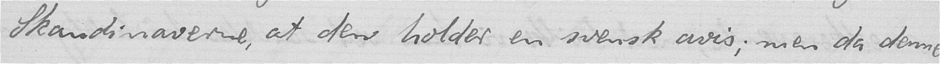Skandinaverne, at den holder en svensk avis; men da denne
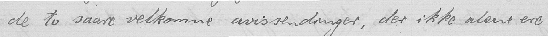de to saare velkomne avissendinger, der ikke alene ere
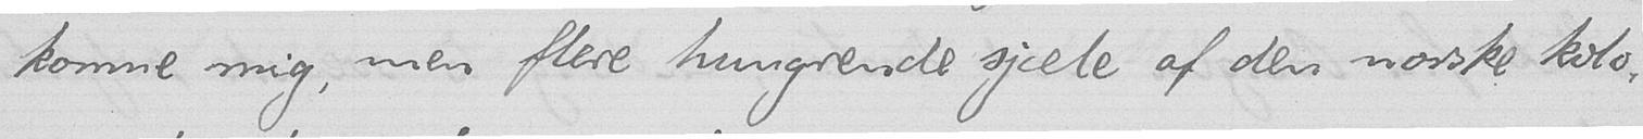komne mig, men flere hungrende sjæle af den norske kolo-
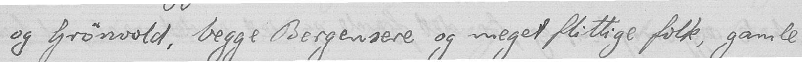og Grønvold, begge Bergensere og meget flittige folk, gamle
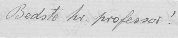Bedste hr professor!
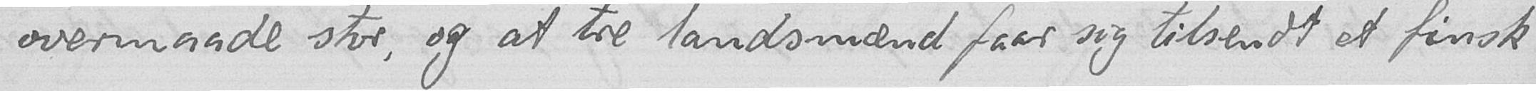overmaade stor, og at tre landsmænd faar sig tilsendt et finsk
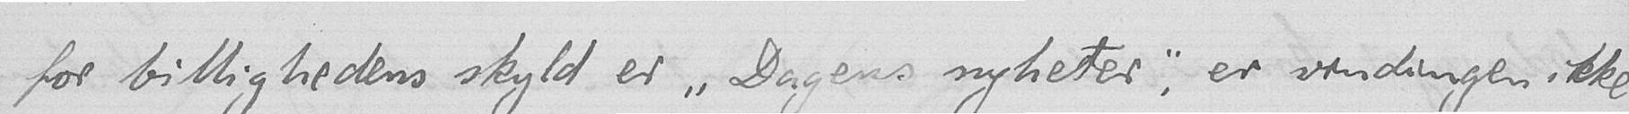for billighedens skyld er "Dagens nyheter", er vindingen ikke
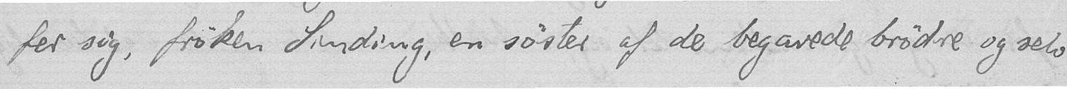fer sig, frøken Sinding, en søster af de begavede brødre og selv
